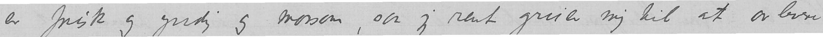er frisk og yndig og morsom, saa jeg rent gruer mig til at avlevere
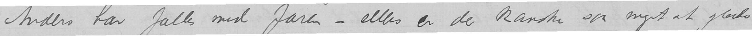Anders har fælles med faren – ellers er der kanske saa meget at glæde
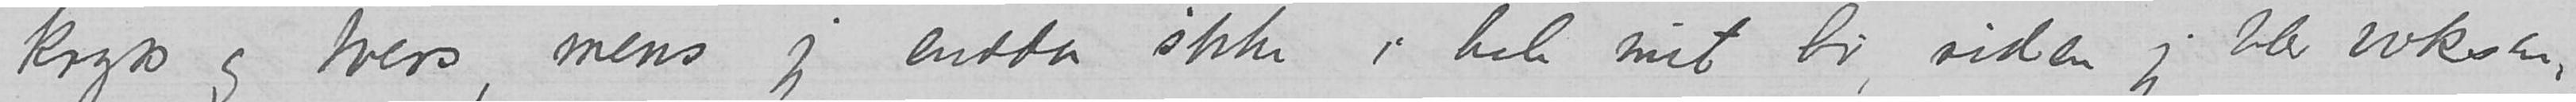kryss og tvers, mens jeg endda ikke i hele mit liv, siden jeg blev voksen,
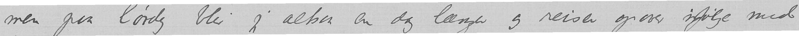men paa lørdag blir jeg altsaa en dag længer og reiser opover ifølge med
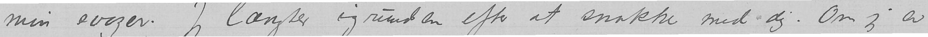min svoger. Jeg længter igrunden efter at snakke med dig. Om jeg er
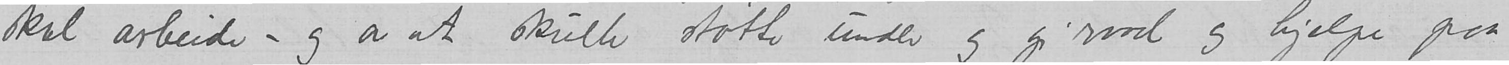skal arbeide – og av at skulle støtte og gi raad og hjelpe paa
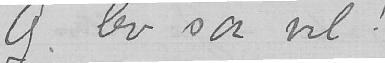Og lev saa vel!
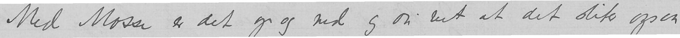Med Mosse er det op og ned og du vet at det sliter ogsaa
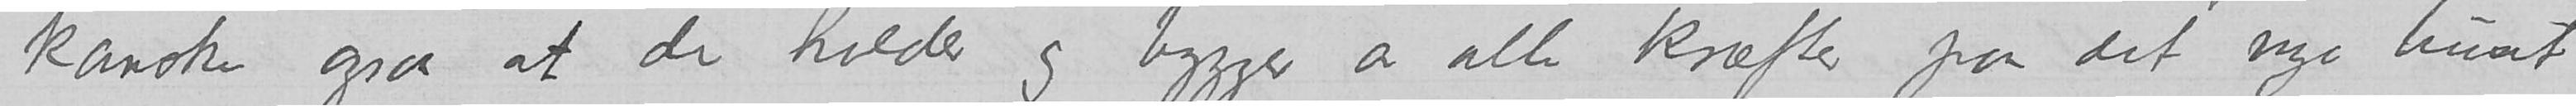kanske ogsaa at de holder og bygger av alle kræfter paa det nye huset
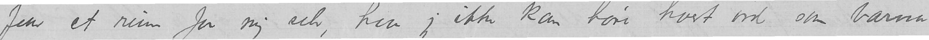faar et rum for mig selv, hvor jeg ikke kan høre hvert ord som barna
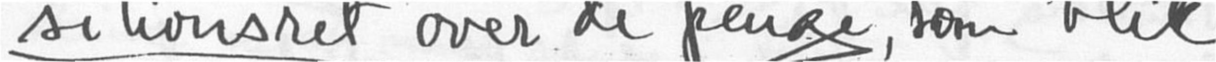sitionsret" over de penge, som blir
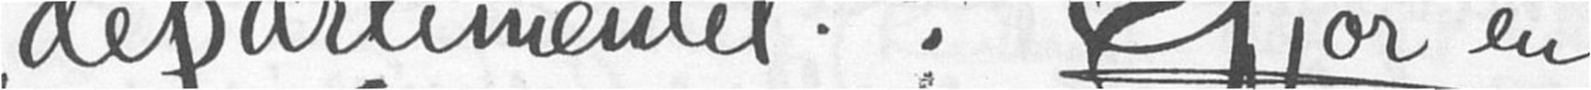"departementet"! Gjør en
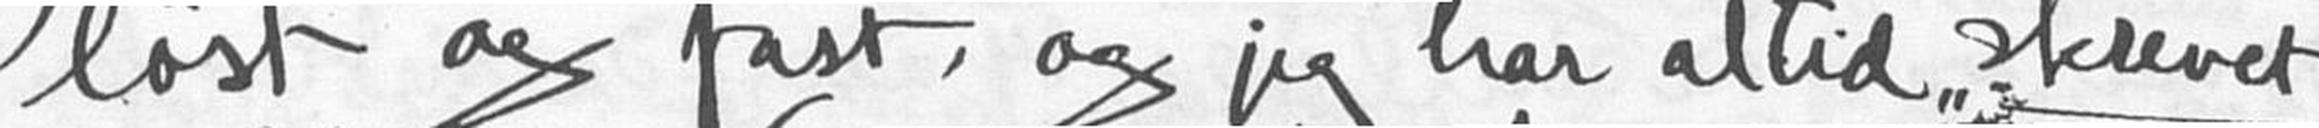løst og fast, og jeg har altid "skrevet"
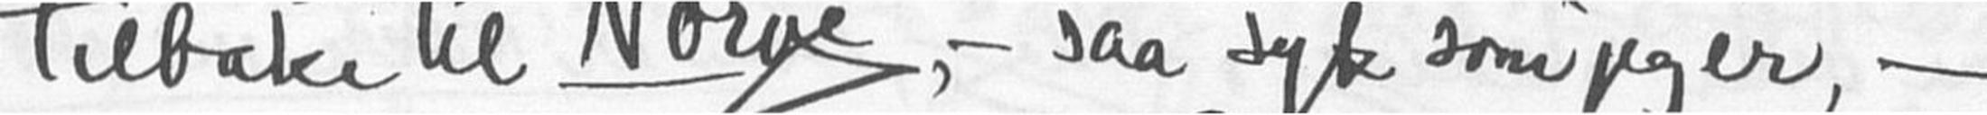tilbake til Norge, - saa syk som jeg er, -
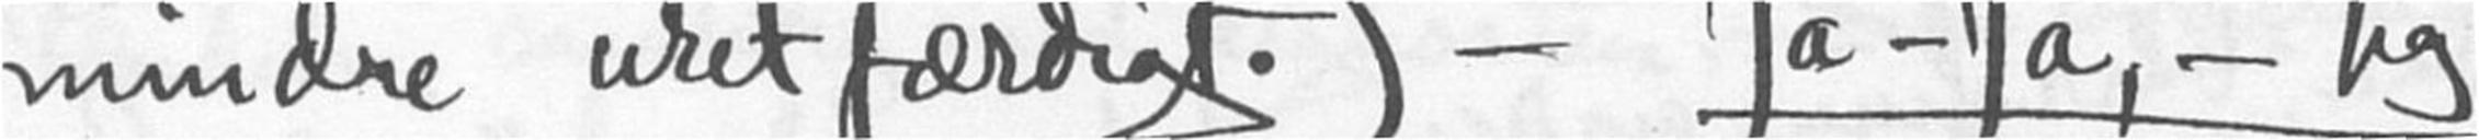mindre uretfærdigt.) Ja - ja, - jeg
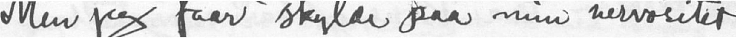Men jeg faar skylde paa min nervøsitet
